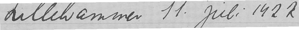Lillehammer 11. juli 1922.
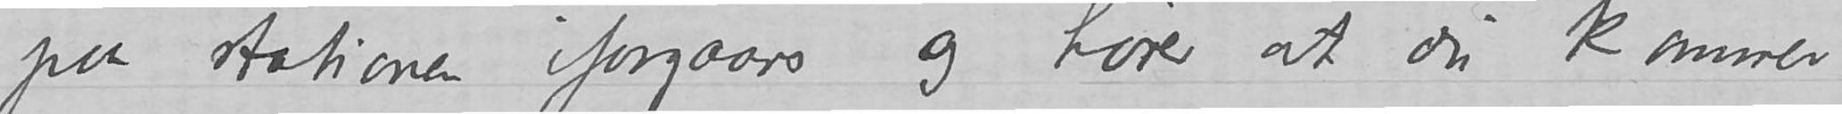paa stationen iforgaars og hører at du kommer
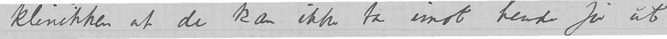klinikken at de kan ikke ta imot hende før ut
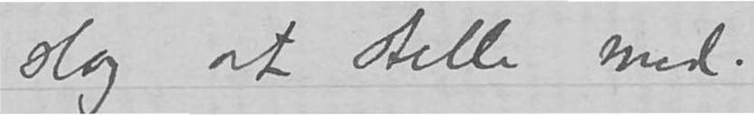slag at stelle med.
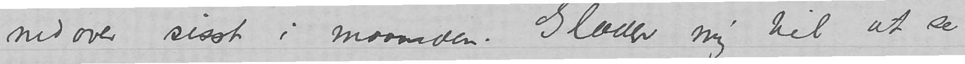nedover sisst i maaneden.  Glæder mig til at se
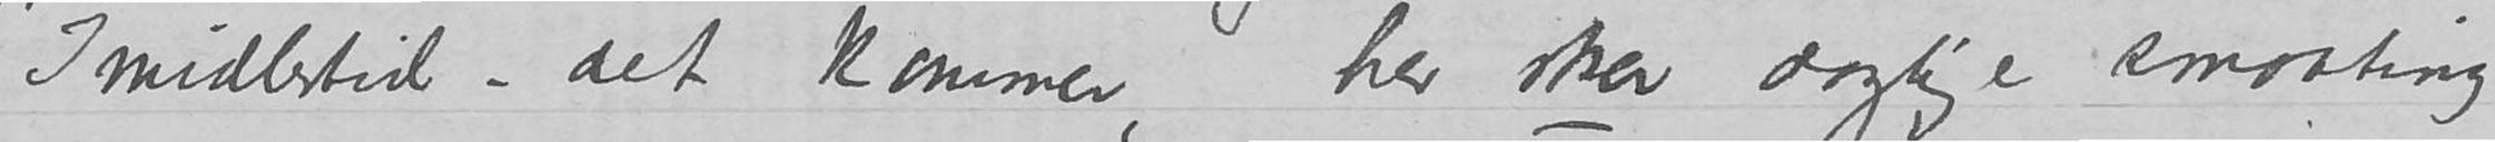Imidlertid – det kommer, her sker daglige smaating
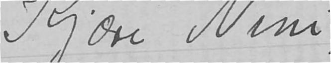Kjære Nini
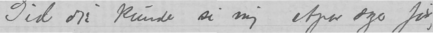Gid du kunde si mig etpar dager før,
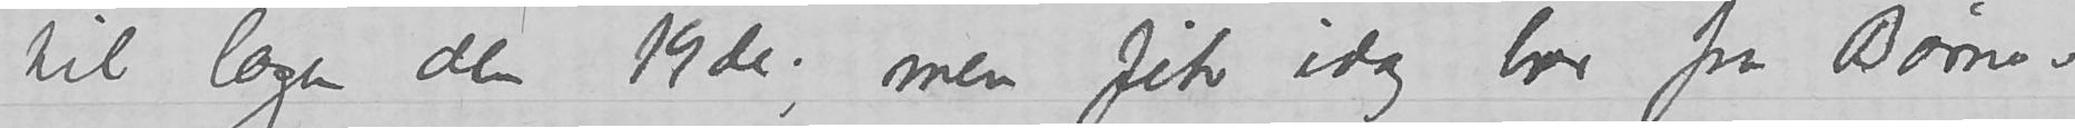til lægen den 19de;., men fik idag brev fra Børne-
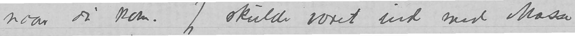naar du kom.  Jeg skulde væoret ind med Mosse
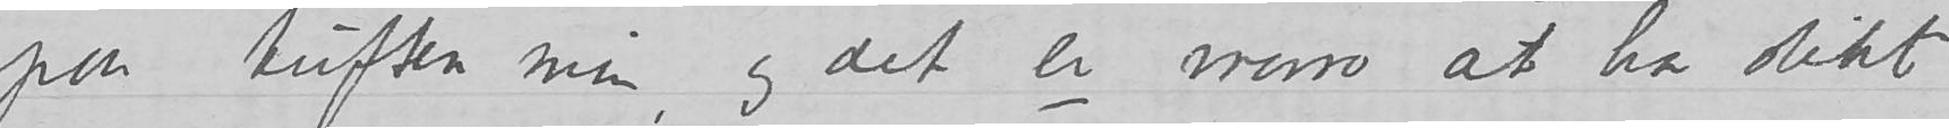paa tuften min, og det er morro at ha slikt
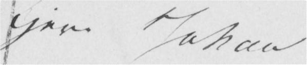Kjere Johan
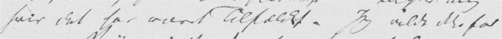hvis det har været Tilfældet. Jeg vilde ikke for
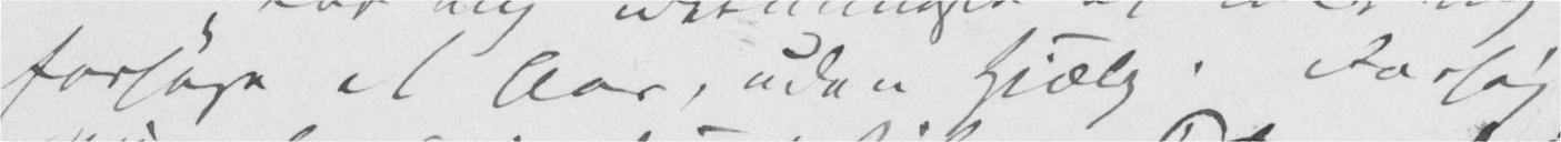forsøge et Aar, uden Hjælp. Forsøg
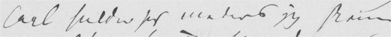Carl sidder her medens jeg skriver
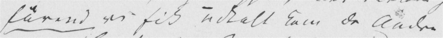førend vi fik udtalt kom de Andre
